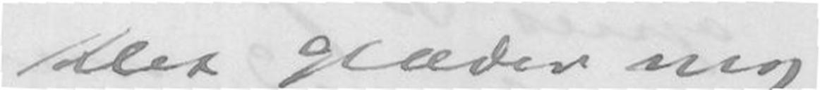Det glæder mig
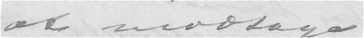at modtage
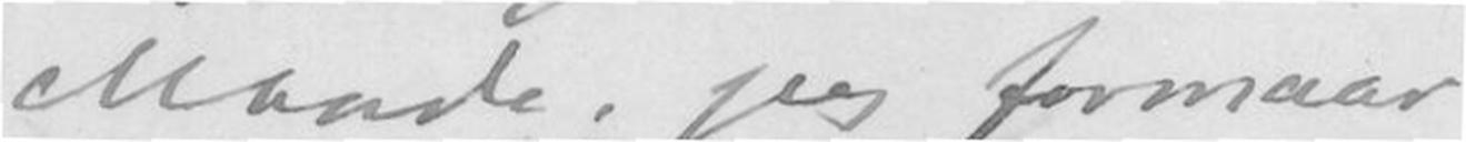Maade, jeg formaar,
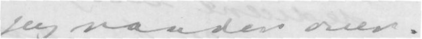jeg raader over.
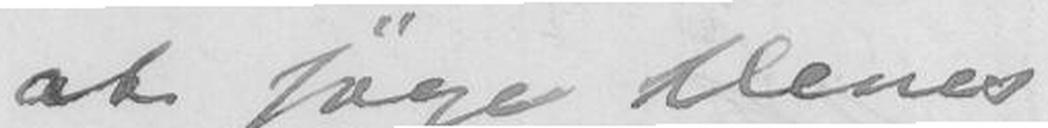at søge Deres
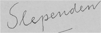Slependen
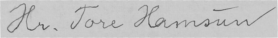Hr. Tore Hamsun
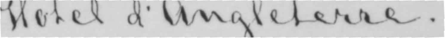Hotel d'Angleterre.
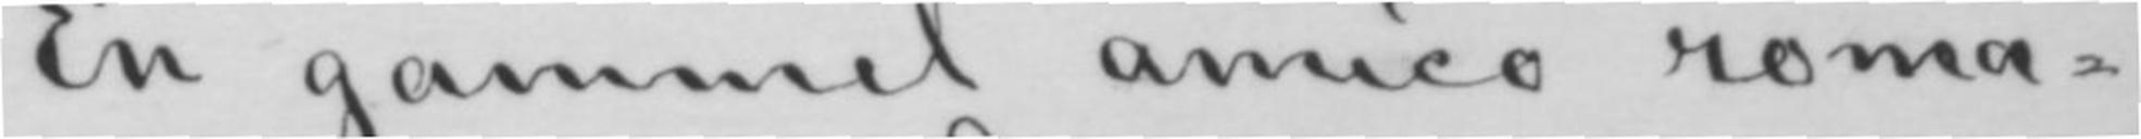En gammel amico roma-
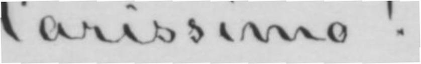Carissimo!
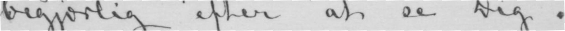begjærlig efter at se Dig.
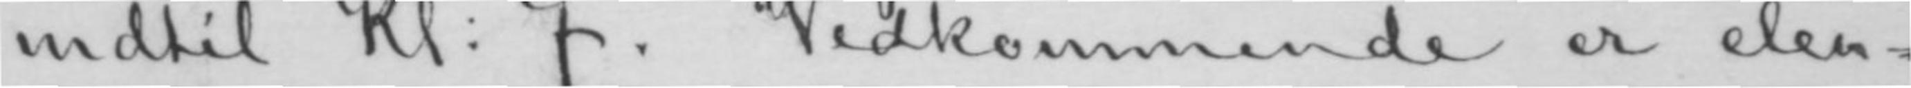indtil Kl: 7. Vedkommende er elen-
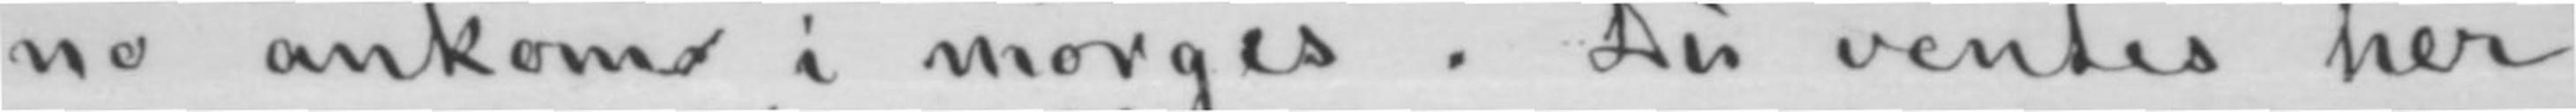no ankom i morges. Du ventes her
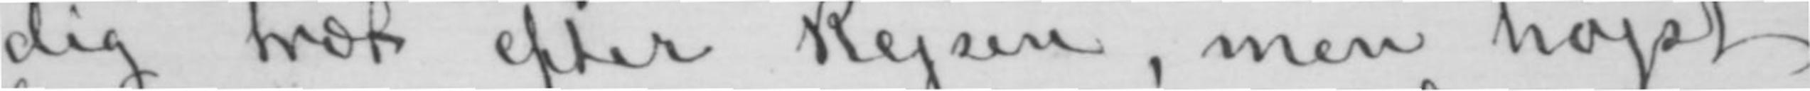dig træt efter Rejsen, men højst
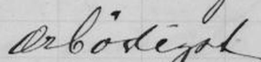ærbødigst
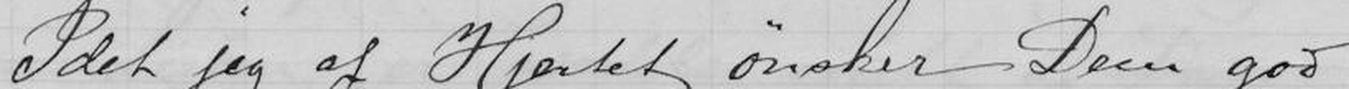Idet jeg af Hjertet ønsker Dem god
